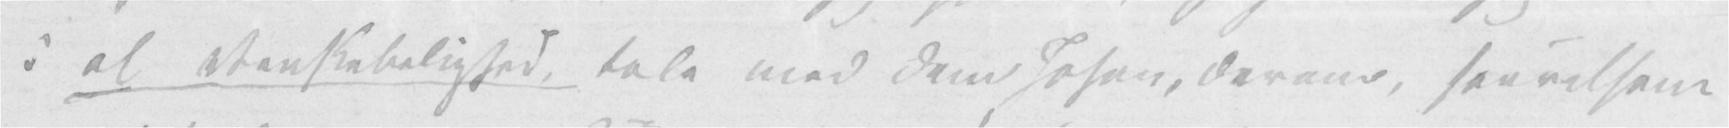i al Venskabelighed tale med Dem Johan, derom, saavelsom
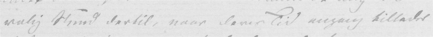rolig Stund dertil, naar Deres Tid engang tillader
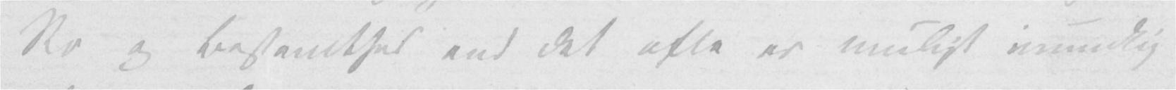Ro og Bestemthed end det ofte er muligt mundtlig
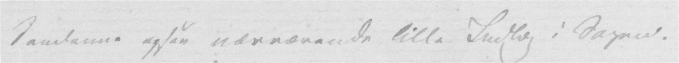Saadanne ogsaa nærværende lille Indlæg i Sagen.
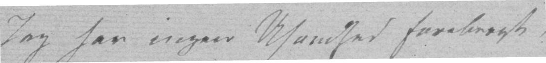Jeg har ingen Usandhed forebragt,
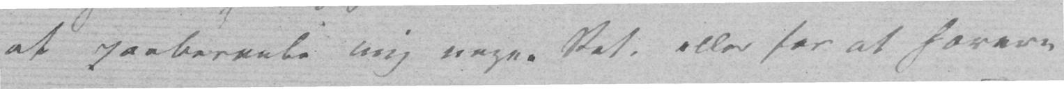at paaberaabe mig nogen Ret, eller for at hovere
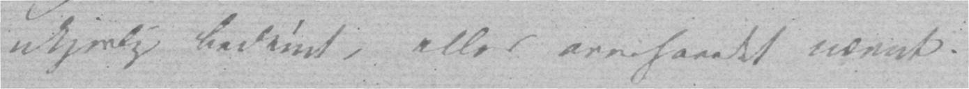ukjerlig bedømt, eller overhovedet nævnt.
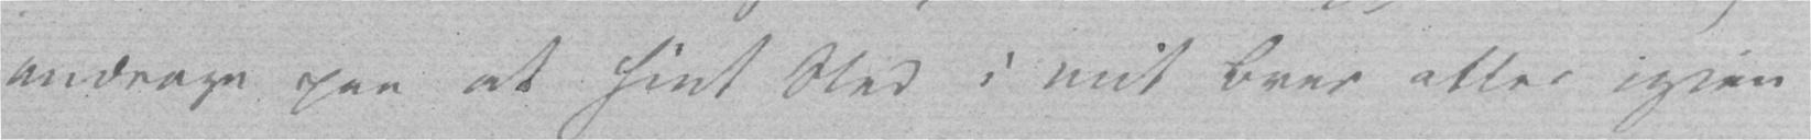andrage paa at hint Sted i mit Brev atter igjen
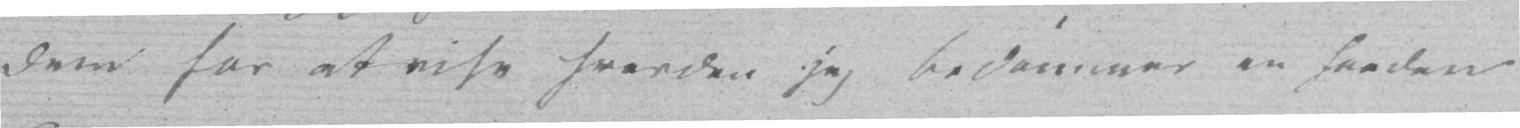Dem for at vise hvordan jeg bedømmer en saadan
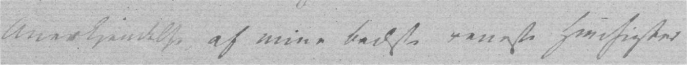Anerkjendelse af mine bedste reneste Hensigter
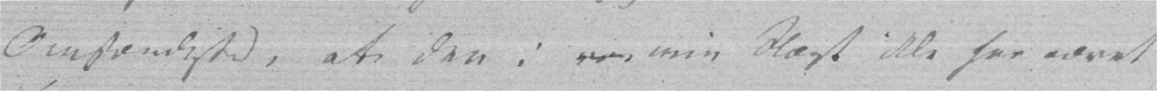Omstændighed, at den i vor min Slægt ikke har været
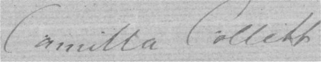Camilla Collett
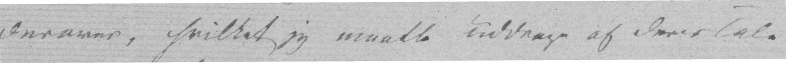derover, hvilket jeg maatte uddrage af Deres Tale
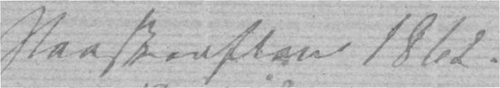Paaskeaften 1862.
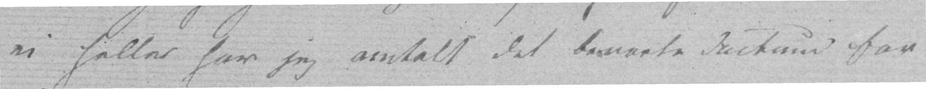ei heller har jeg omtalt det bevarte Factum for
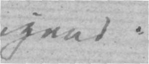igaar.
